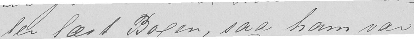ler læst Bogen, saa ham var
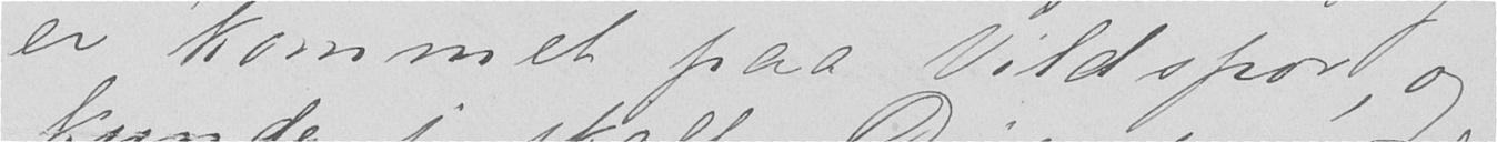er kommet paa Vildspor, og
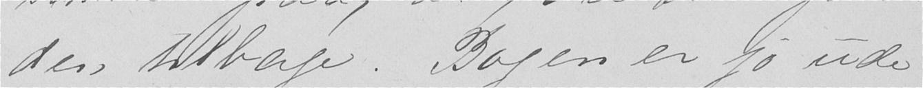den tilbage. Bogen er jo ude
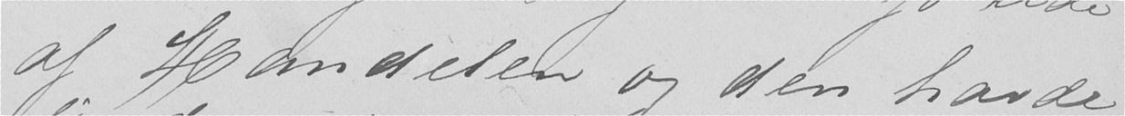af Handelen og den havde
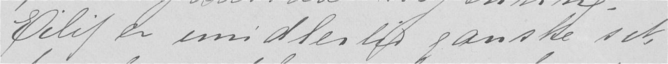Eilif er imidlertid ganske sik-
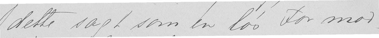dette sagt som en løs Formod-
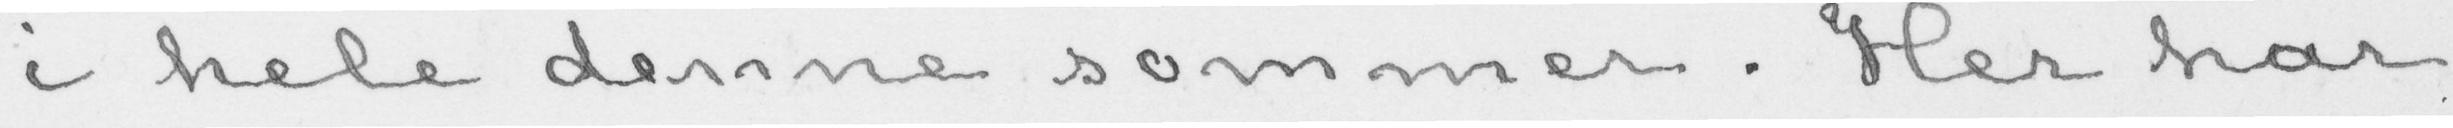i hele denne sommer. Her har
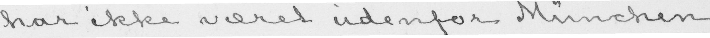har ikke været udenfor München
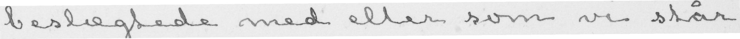beslægtede med eller som vi står
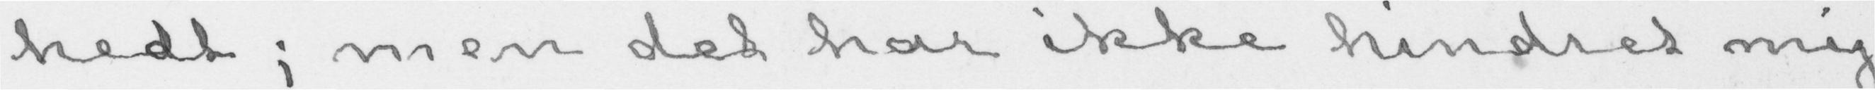hedt; men det har ikke hindret mig
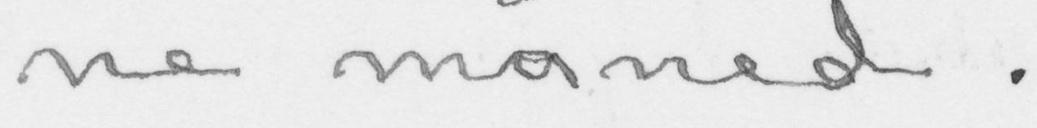ne måned.
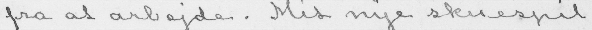fra at arbejde. Mit nye skuespil,-
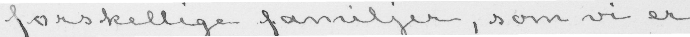forskellige familjer, som vi er
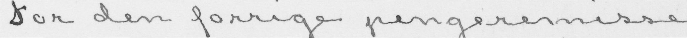For den forrige pengeremisse
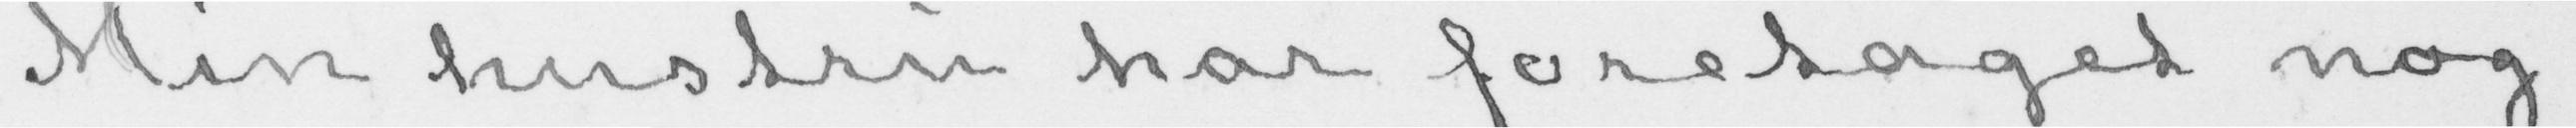Min hustru har foretaget nog-
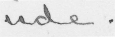ude.
